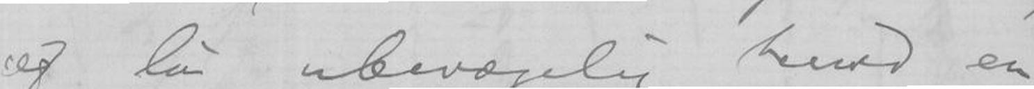og lå ubevægelig herved en
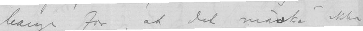bange for, at det måske ikke
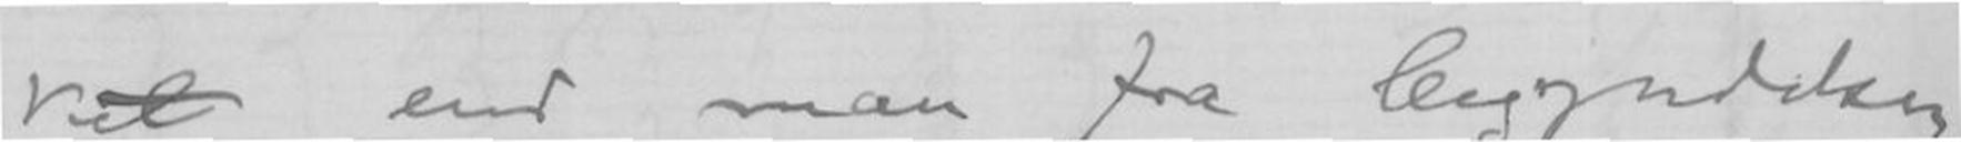ket end man fra begyndelsen
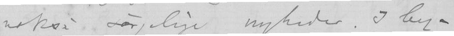nokså sørgelige nyheder. I beg.
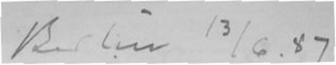Berlin 13/6.87
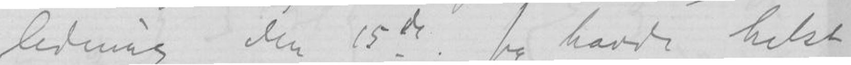ledning den 15de. Jeg havde helst
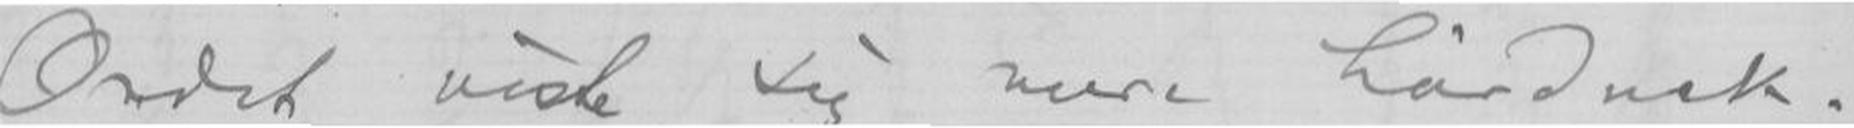Ordet viste sig mere hårdnak-
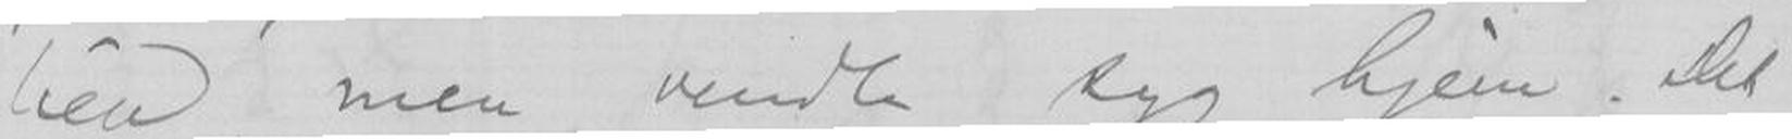lien men vendte syg hjem. Det
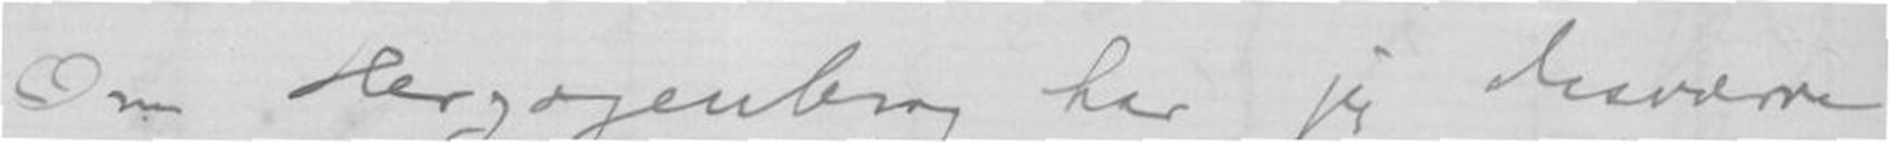Om Herzogenberg har jeg desværre
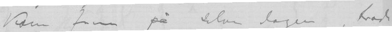kom frem på selve dagen, trode
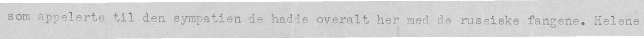som appelerte til den sympatien de hadde overalt her med de russiske fangene. Helene
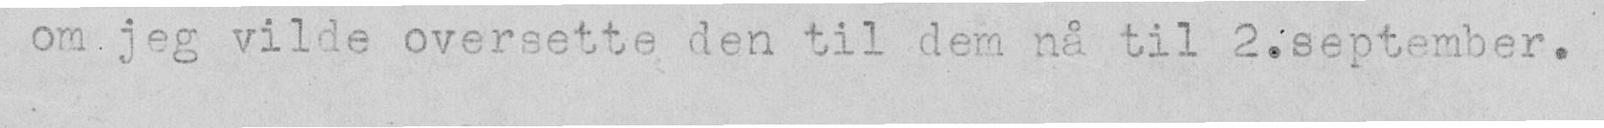om jeg vilde oversette den til dem nå til 2.september.
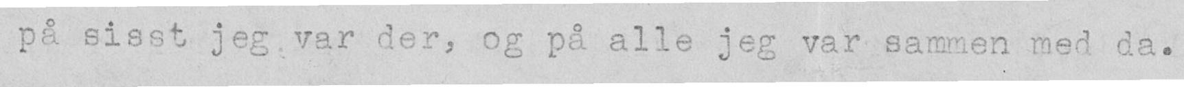på sisst jeg var der, og på alle jeg var sammen med da.
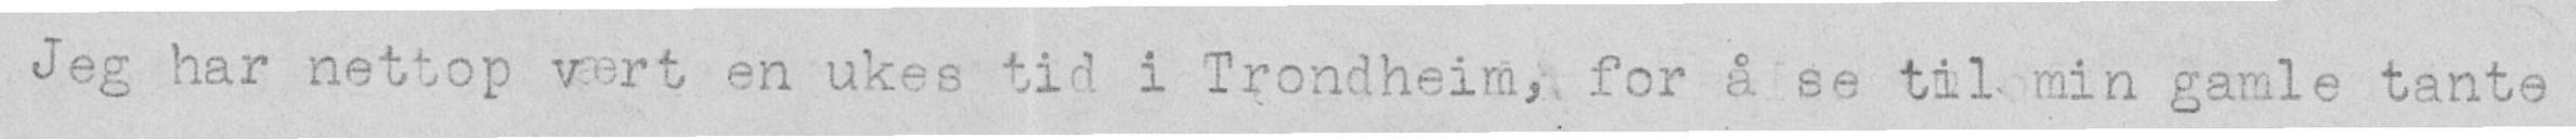Jeg har nettop vært en ukes tid i Trondheim, for å se tilemin gamle tante
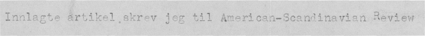Innlagte ärtikel,skrev jeg til American-Scandinavian Review
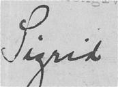Sigrid
